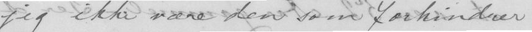jeg ikke være den som forhindrer
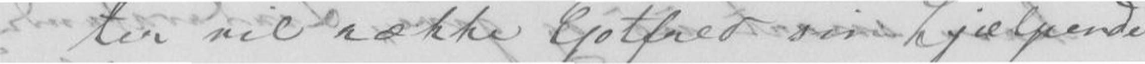ter vil række Gotfred sin hjælpende
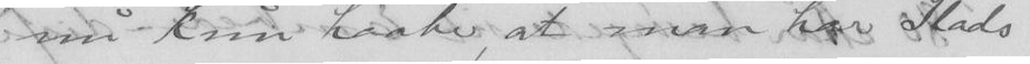nu kun haabe, at man har Plads
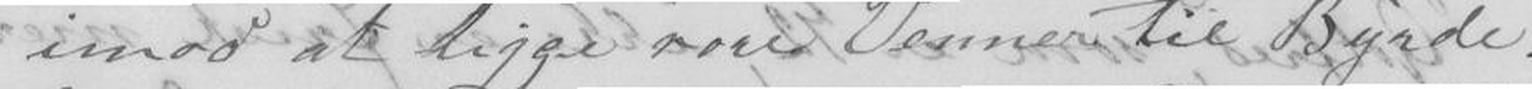imod at ligge vore Venner til Byrde.
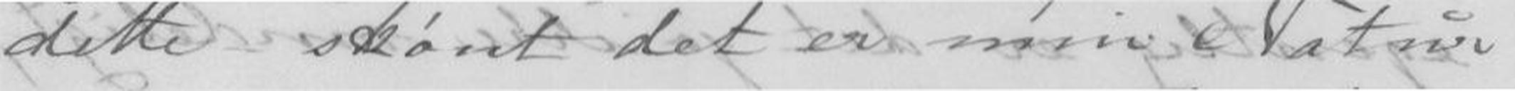dette skønt det er mine Natur
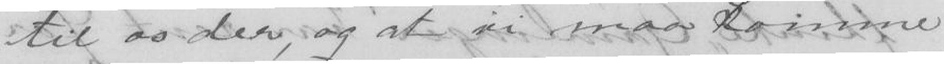til os der, og at vi maa Komme
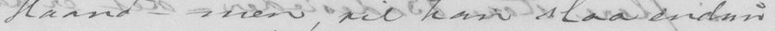Haand - men; vil han slaa endnu
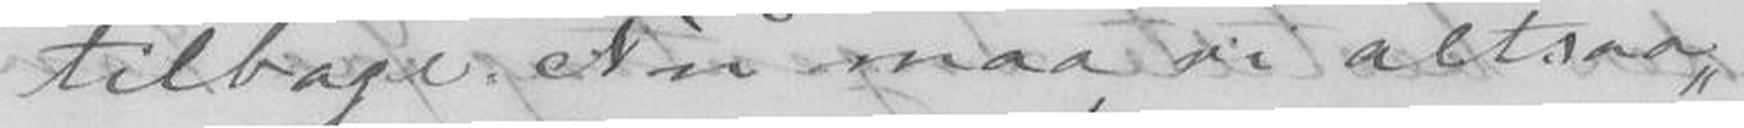tilbage. Nu maa vi altsaa
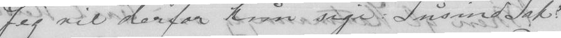Jeg vil derfor kun sige: Tusind Tak!
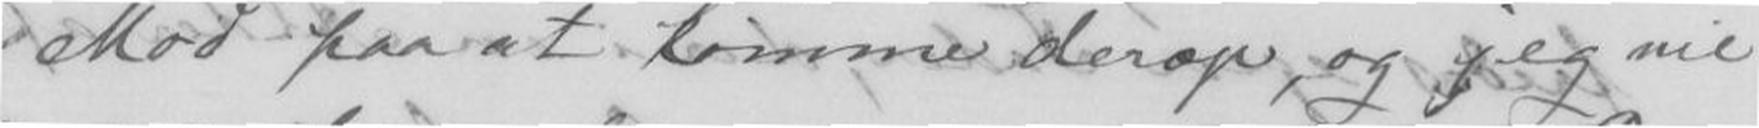Mod paa at komme derop, og jeg vil
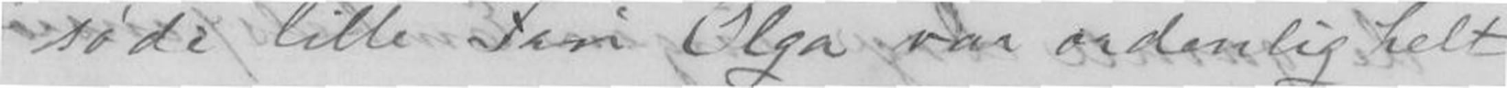søde lille Fru Olga var ordentlig helt
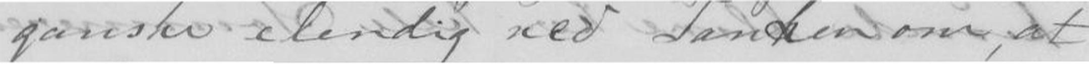ganske elendig ved Tanken om, at
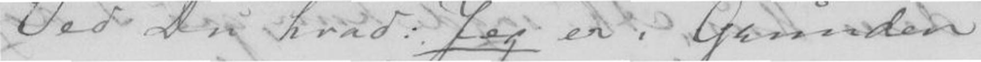Ved Du hvad: Jeg er i Grunden
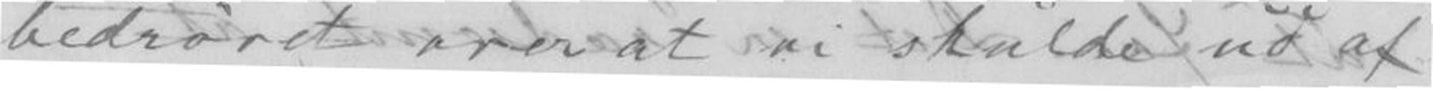bedrøvet over at vi skulde ud af
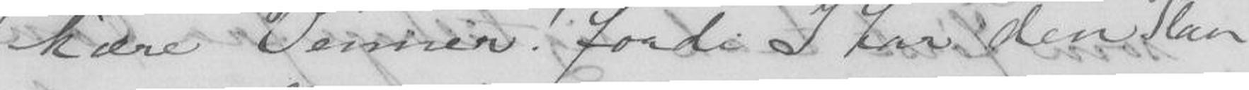kære Venner! fordi I har den Plan
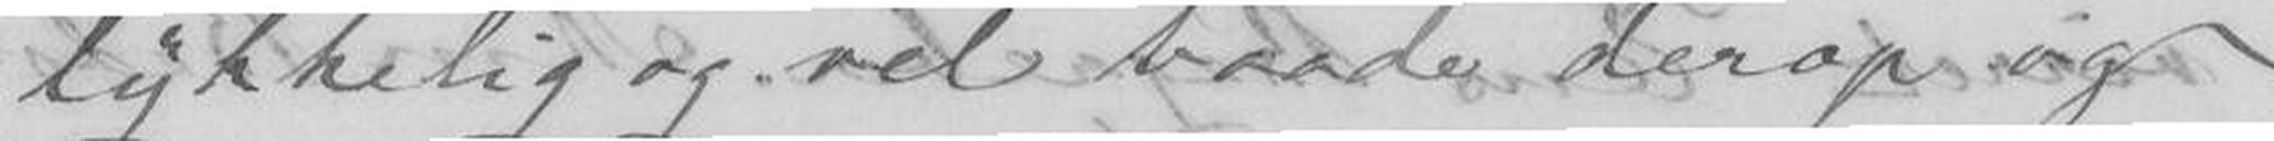lykkelig og vel baade derop og
